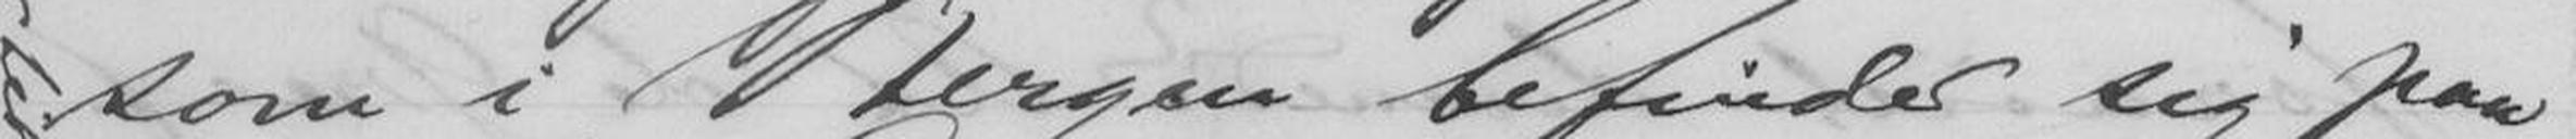som i Bergen befinder sig paa
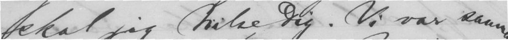skal jeg hilse Dig. Vi var sammen
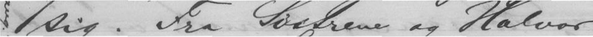sig. Fra Søstrene og Halvor
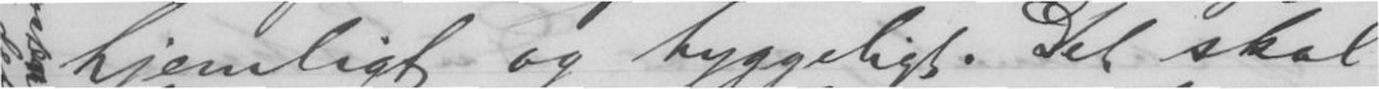hjemligt og hyggeligt. Det skal
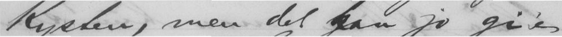Kysten, men det kan jo gi'e
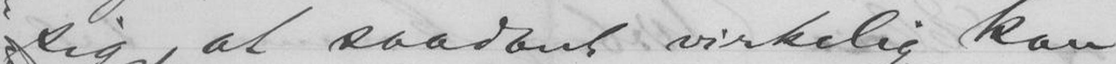sig, at saadant virkelig kan
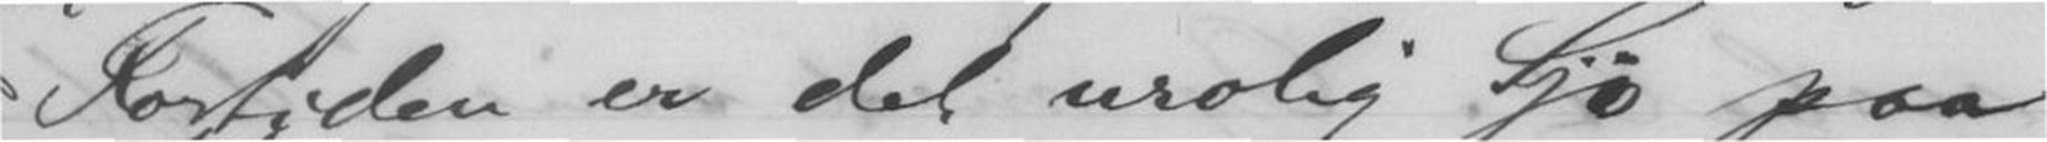Fortiden er det urolig Sjø paa
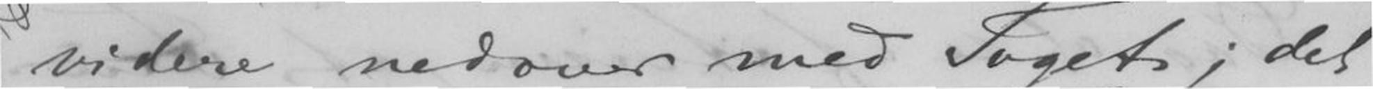videre nedover med Toget; det
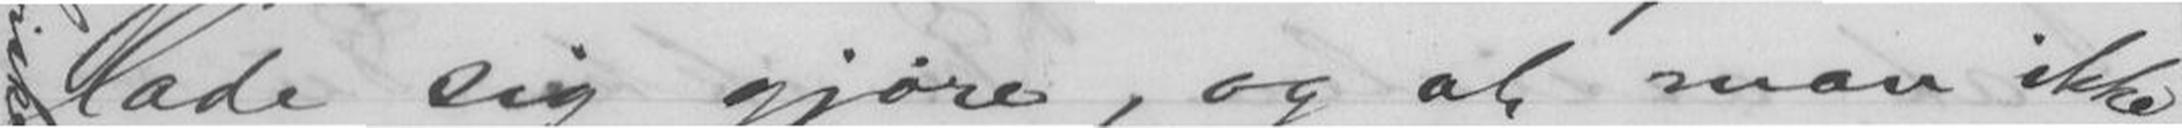lade sig gjøre, og at man ikke
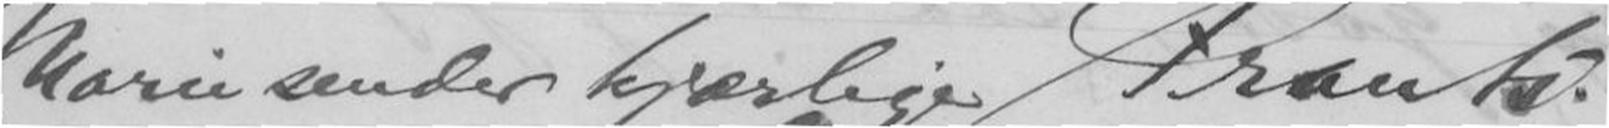Marie sender kjærlig Frants
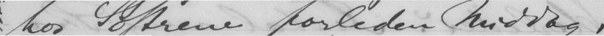hos Søstrene forleden Middag,
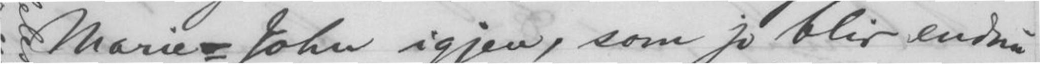Marie=John igjen, som jo blir endnu
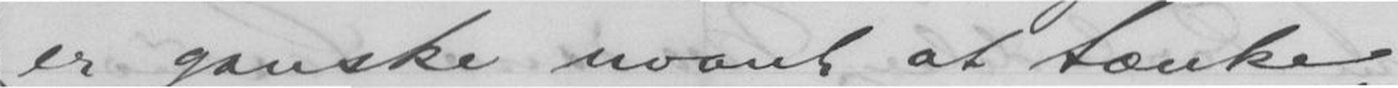er ganske uvant at tænke
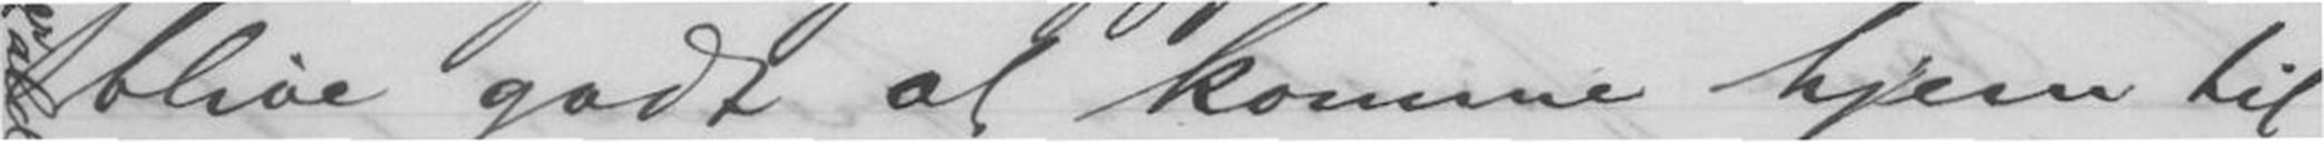blive godt at komme hjem til
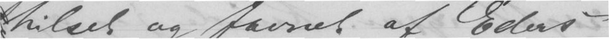hilset og farvel af Eders
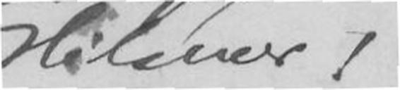Hilsener!
